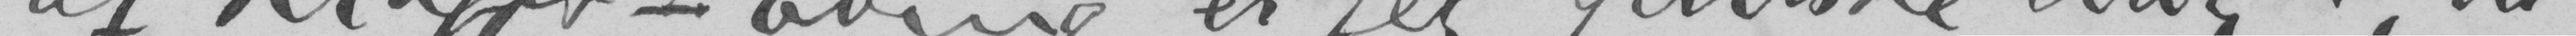af Krafft-Ebing er jeg ganske enig i, at
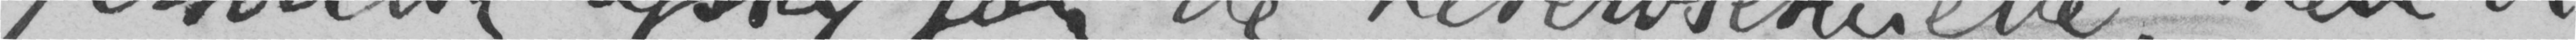personlig afsky for de heterosexuelle; men vi
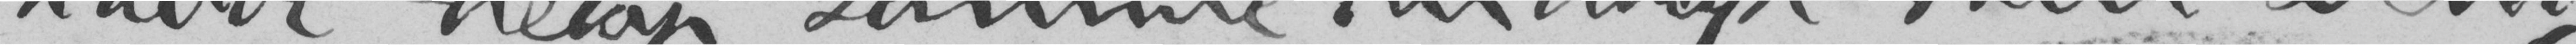havde netop samme indtryk som Dem,
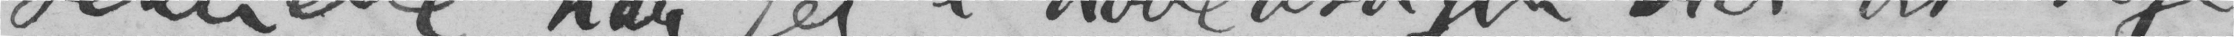sexuelle har jeg i hovedsagen blot at sige,
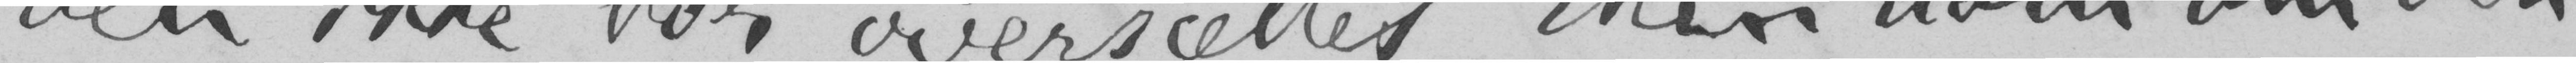den ikke bør oversættes. Min dom om den
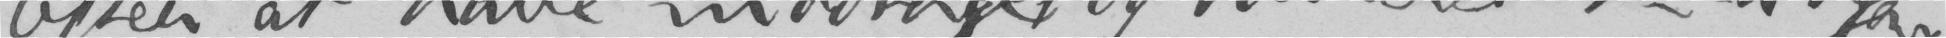Efter at have modtaget og studeret 7de udgave
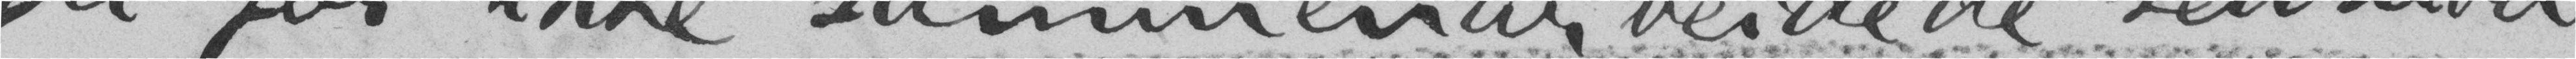fri for ikke sammenarbeidede selvmod-
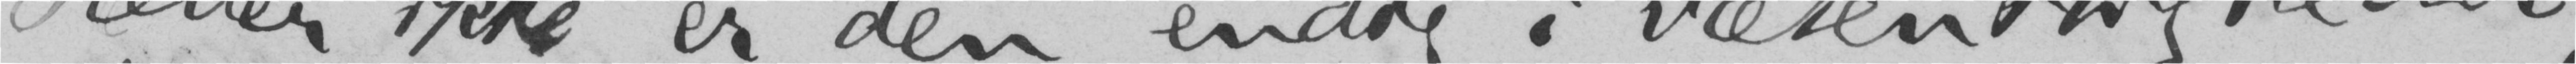Heller ikke er den, endog i væsentligheder,
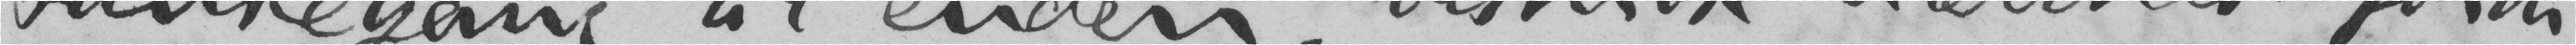tankegang til enden, vistnok nærmest fordi
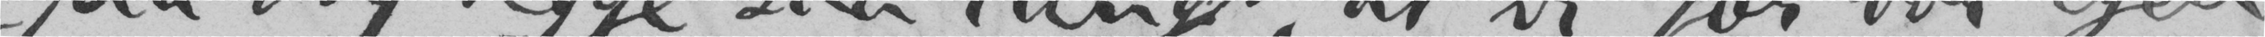gaa dog begge saa langt, at vi for vor egen
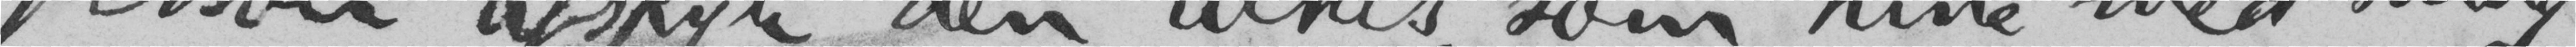person afskyr den actus, som hine med smag
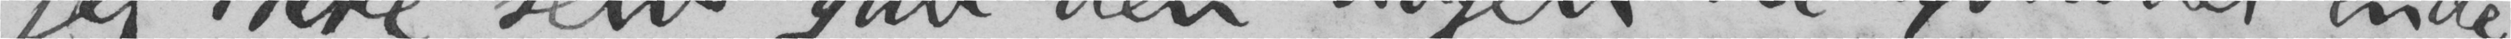jeg ikke selv gav den nogen vel afsluttet ende.
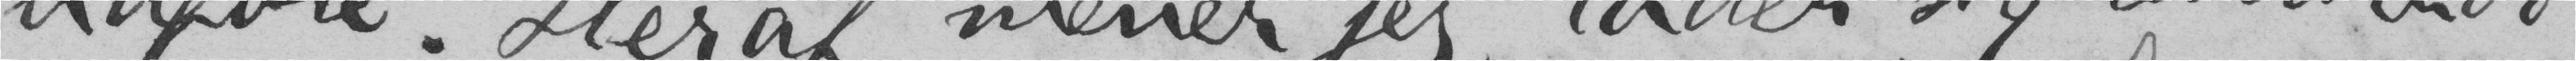udføre. Heraf, mener jeg, lader sig omvendt
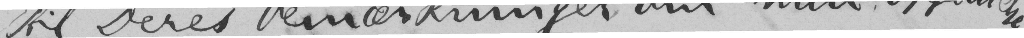Til Deres bemærkninger om min opfattelse
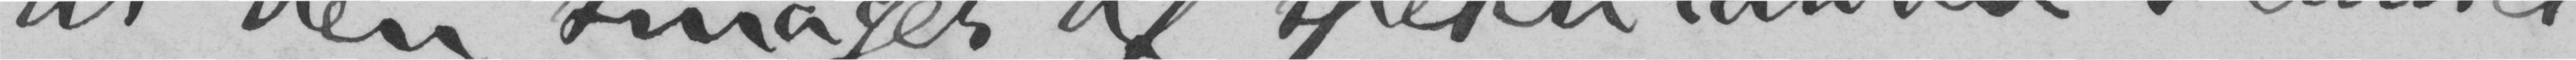at den smager af spekulation i emnet.
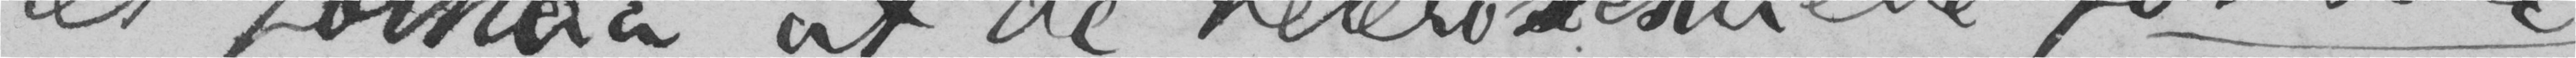let forstaa, at de heterosexuelle for sine
